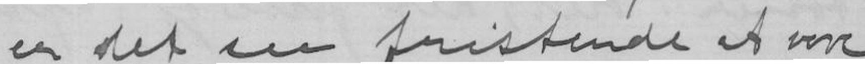er det nu fristende at vove
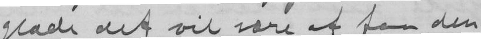glæde det vil være at faa den,
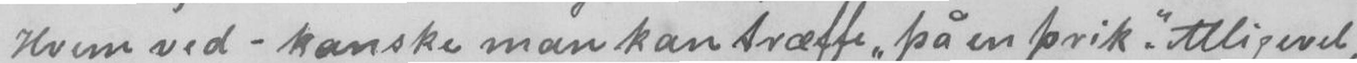Hvem ved - kanske man kan træffe på en prik. Alligevel,
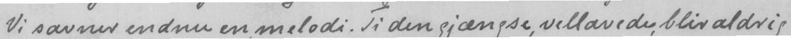Vi savner endnu en melodi. Tiden gjængse, vellavede, blir aldrig
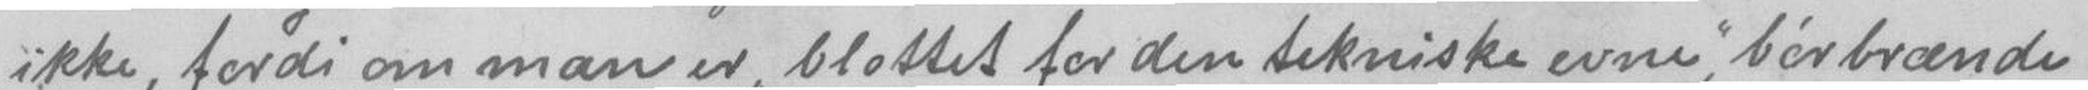ikke, fordi om man er "blottet for den tekniske evne", bør brænde
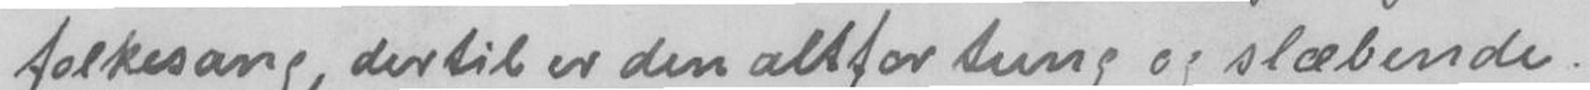folkesang, dertil er den altfor tung og slæbende.
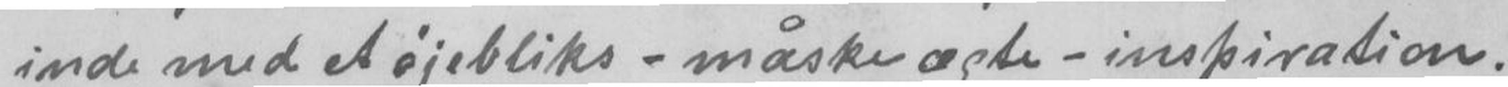inde med et øjebliks - måske ægte - inspiration.
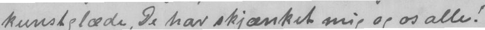kunstglæde, De har skjænket mig og os alle!
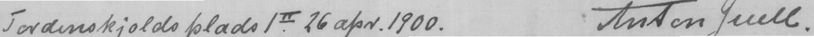Tordenskjoldsplads 1II 26 apr. 1900. Anton Juell.
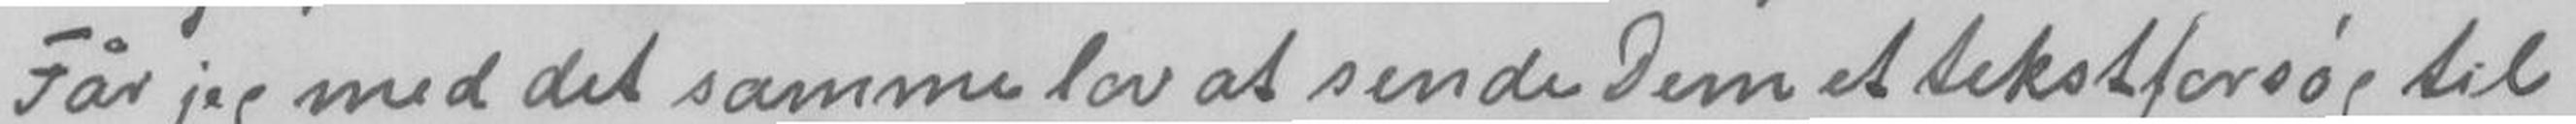Får jeg med det samme lov at sende Dem et tekstforsøg til
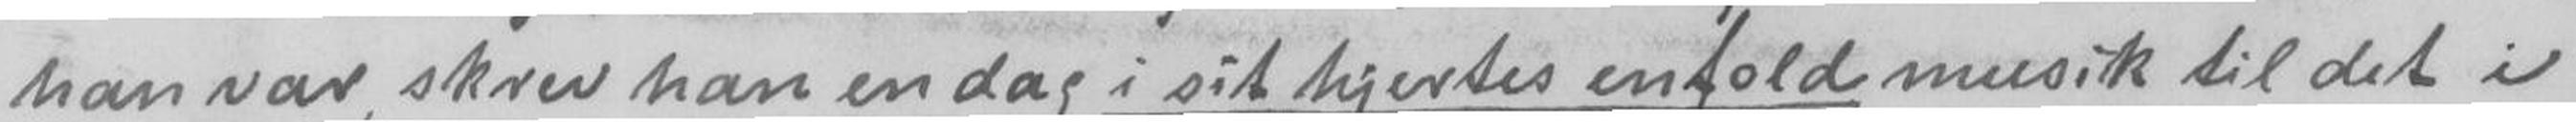han var, skrev han en dag i sit hjertes enfold musik til det i
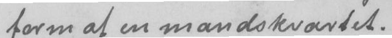form af en mandskvartet.
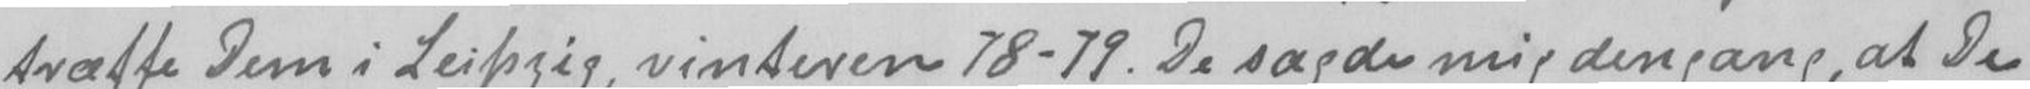træffe Dem i Leipzig, vinteren 78-79. De sagde mig dengang, at De
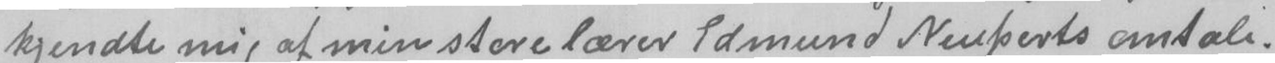kjendte mig af min store lærer Edmund Neuperts omtale.
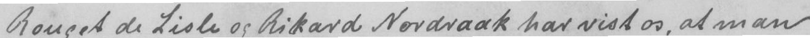Rouget de Lisle og Rikard Nordraak har vist os, at man
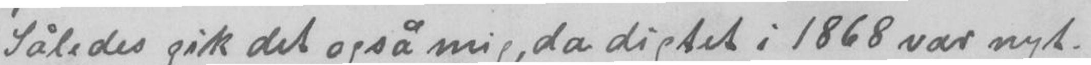Således gik det også mig, da digtet i 1868 var nyt.
